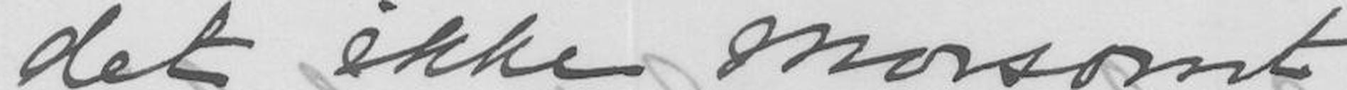det ikke morsomt
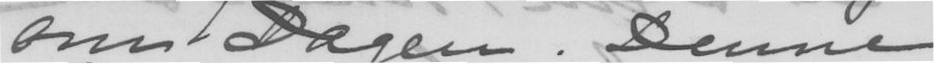om Dagen. Denne
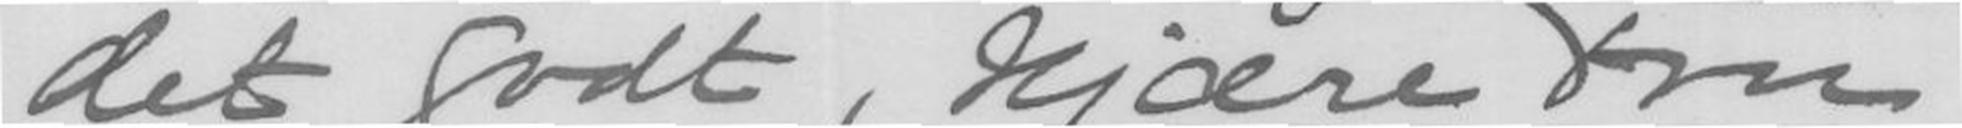det godt, kjære Fru
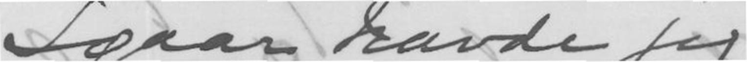Igaar havde jeg
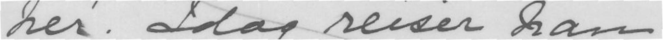her. Idag reiser han
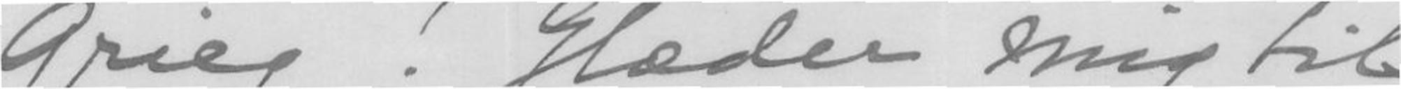Grieg! Glæder mig til
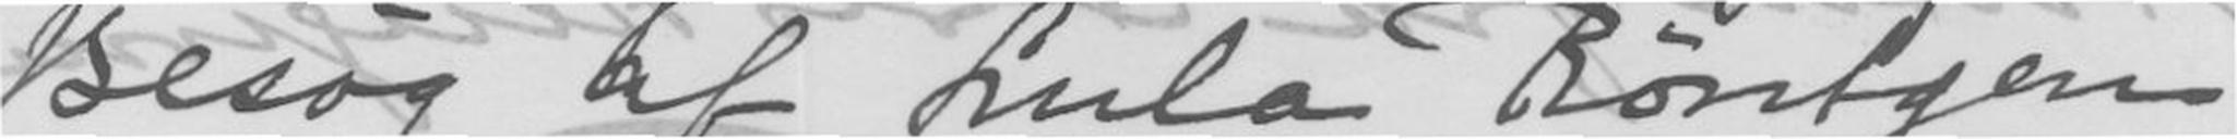Besøk af Lula Røntgen,
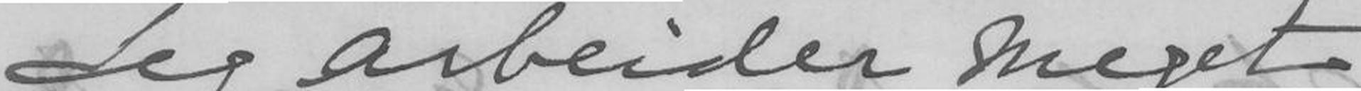Jeg arbeider meget
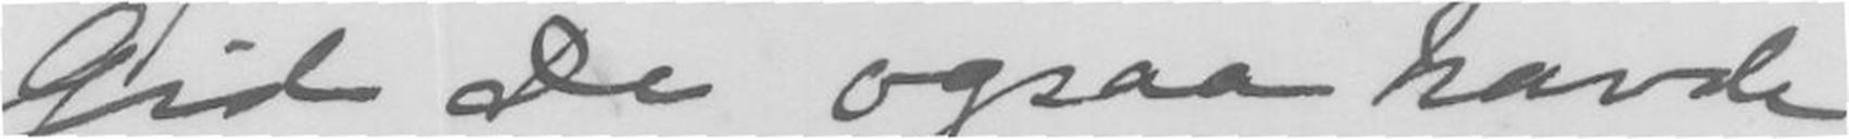Gid de ogsaa havde
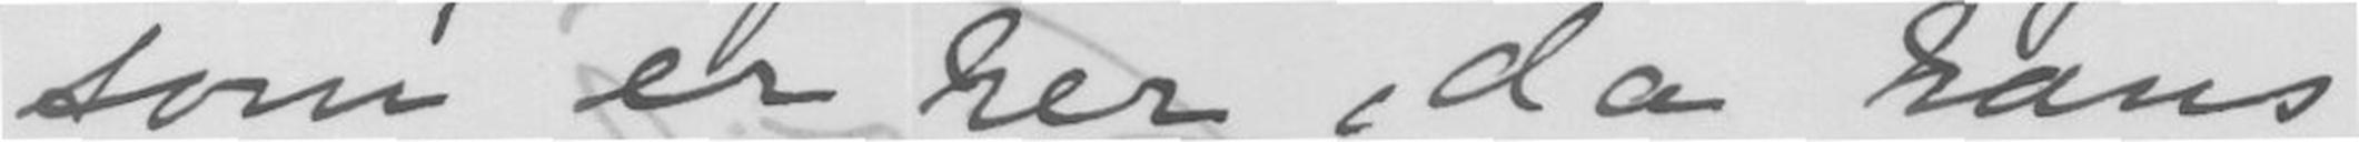som er her, da hans
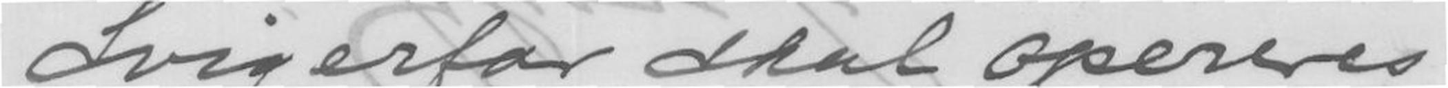Svigerfar skal opereres
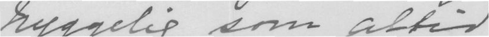hyggelig som altid
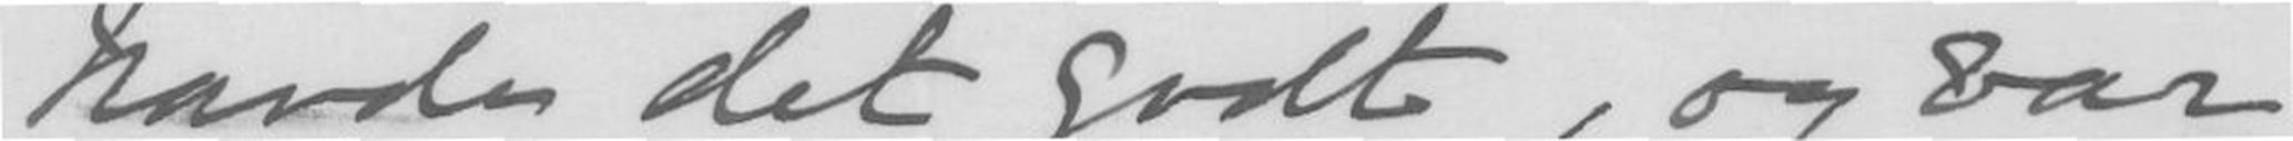havde det godt, og var
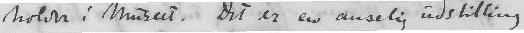holder i museet. Det er en anselig udstilling
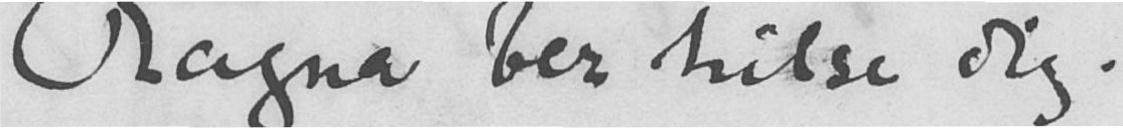Ragna ber hilse dig.
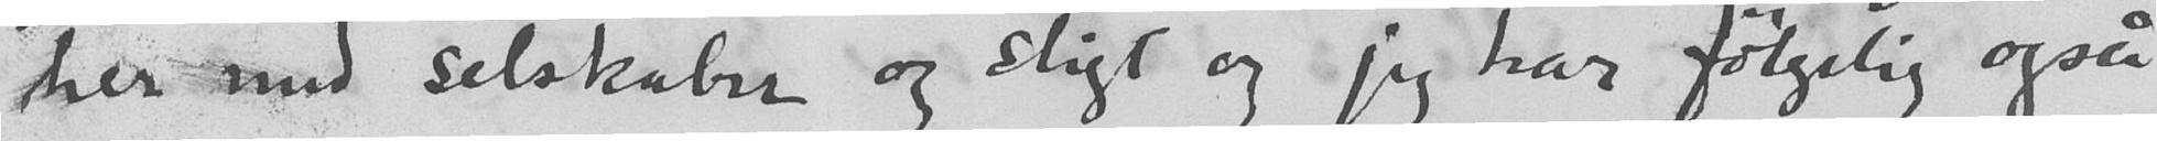her med selskaber og sligt og jeg har følgelig også
